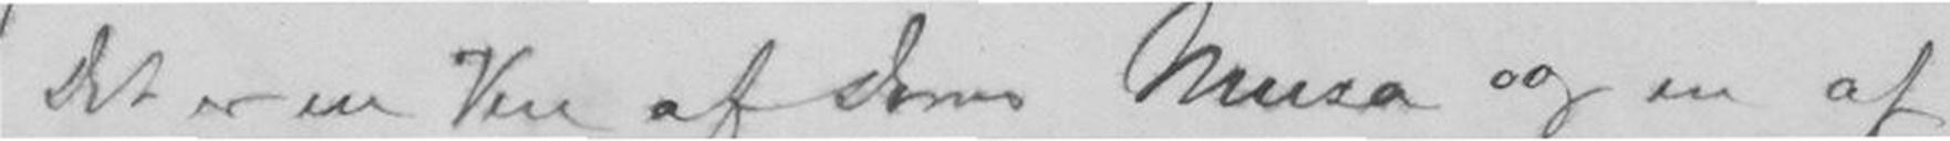Det er en Ven af Deres Musa og en af
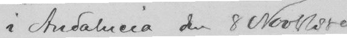i Andalucia den 8 Novb 1880
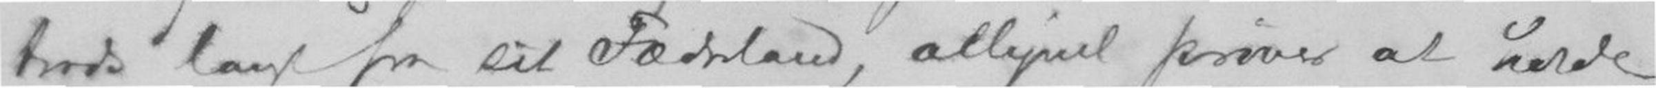trods langt fra sit Fædreland, alligevel prøver at holde
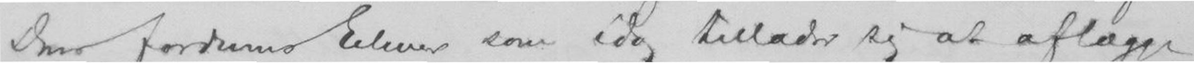Deres fordums Elever som idag tillader sig at aflægge
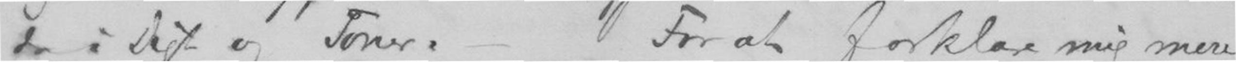de i Digt og Toner.For at forklare mig mere
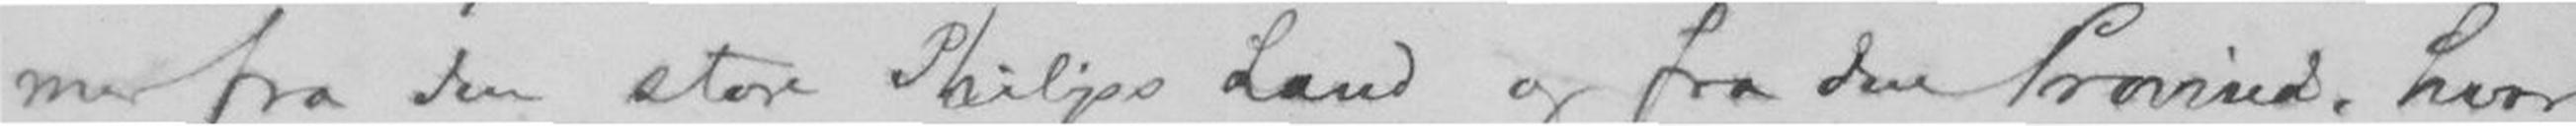mer fra den store Philips Land og fra den Provinds, hvor
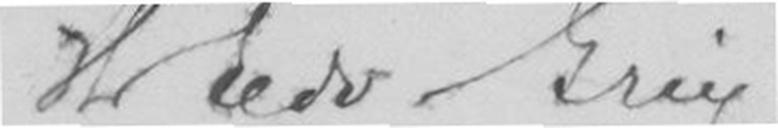Her Edv Grieg
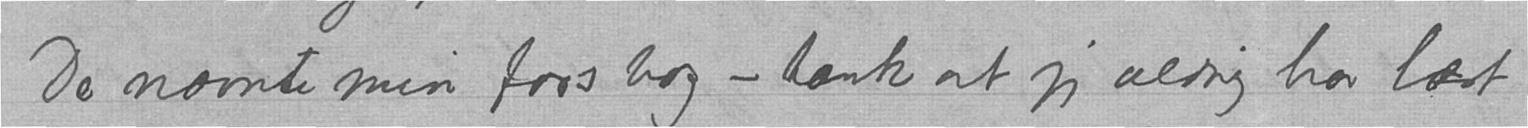De nævnte min fars bog – tænk at jeg aldrig har læst
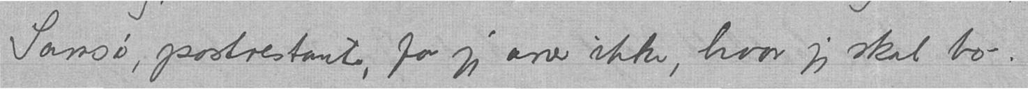Samsø, postrestante, for jeg aner ikke, hvor jeg skal bo-.
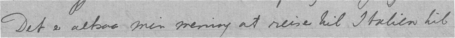Det er altsaa min mening at reise til Italien til
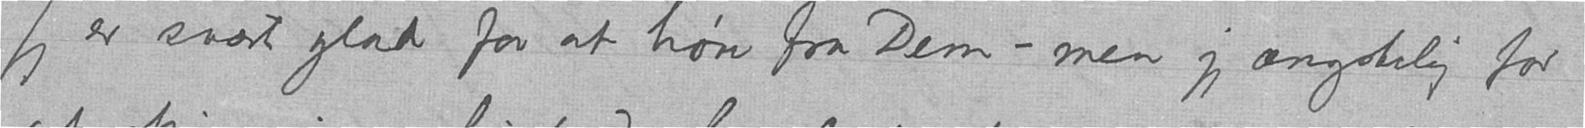Jeg er svært glad for at høre fra Dem - men jeg ængstelig for
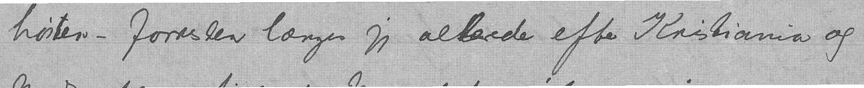høsten - forresten længes jeg allerede efter Kristiania og
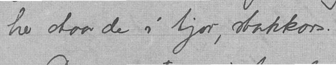her staar de i tjor, stakkars.
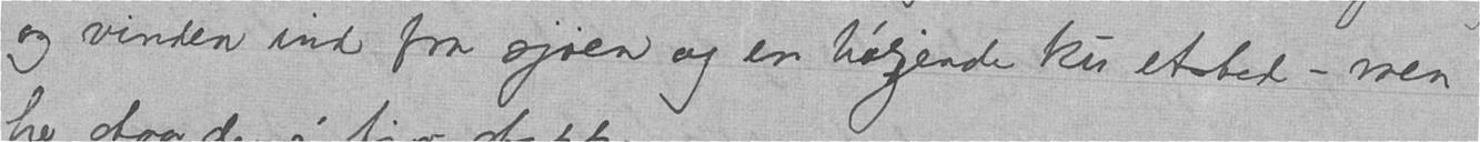og vinden ind fra sjøen og en ende ku etsted – men
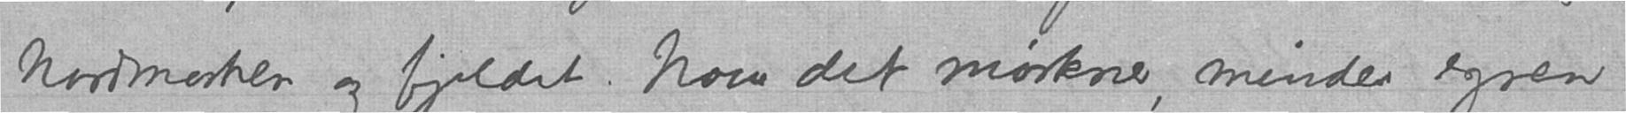Nordmarken og fjeldet. Naar det mørkner, minder egnen
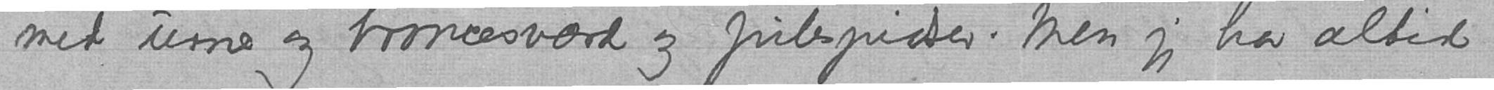med urne og broncesværd og pilespidser. Men jeg har altid
